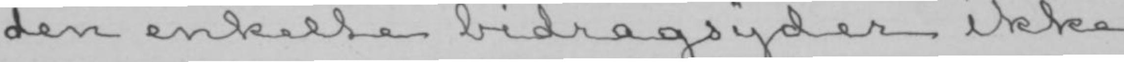den enkelte bidragsyder ikke
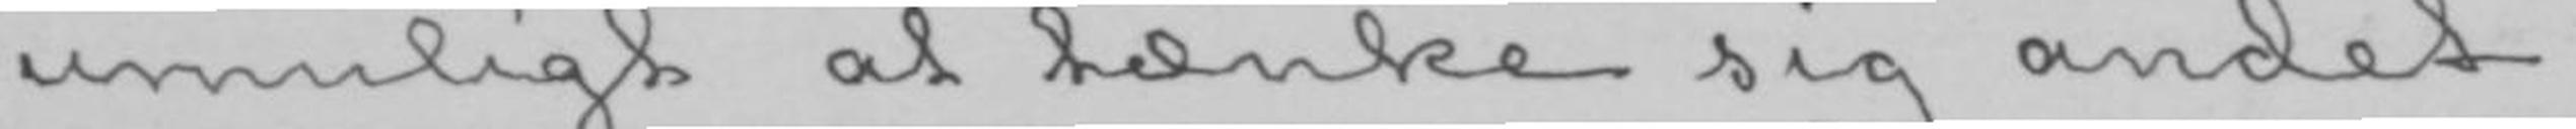umuligt at tænke sig andet.
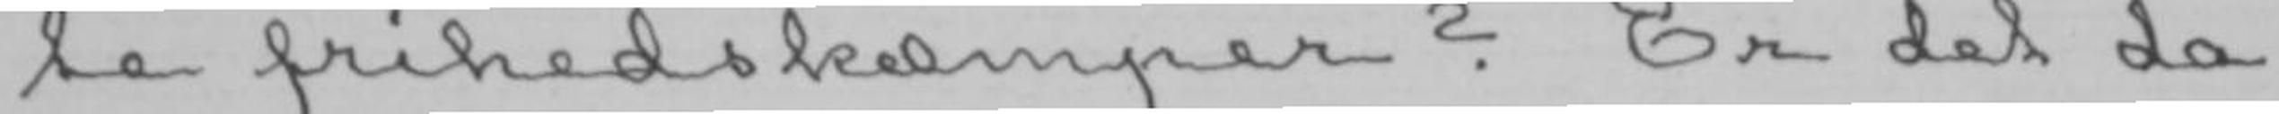te frihedskæmper? Er det da
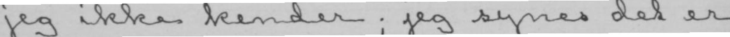jeg ikke kender; jeg synes det er
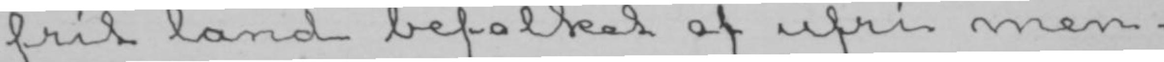frit land befolket af ufri men-
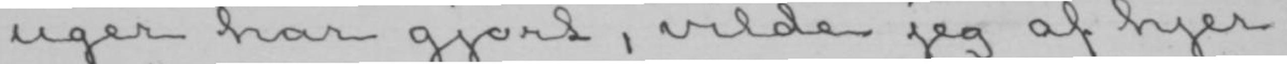uger har gjort, vilde jeg af hjer-
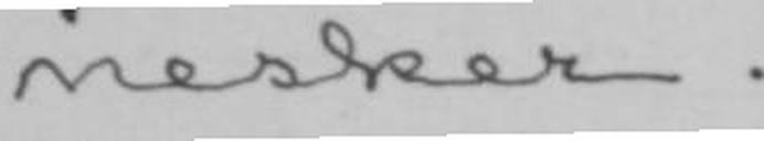nesker.
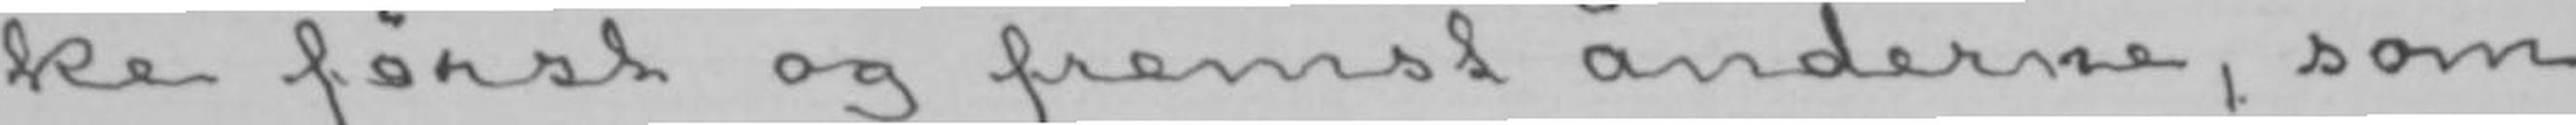ke først og fremst ånderne, som
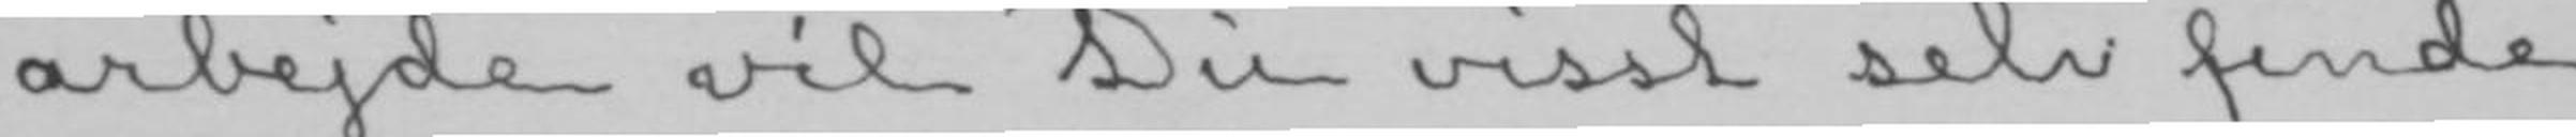arbejde vil Du visst selv finde
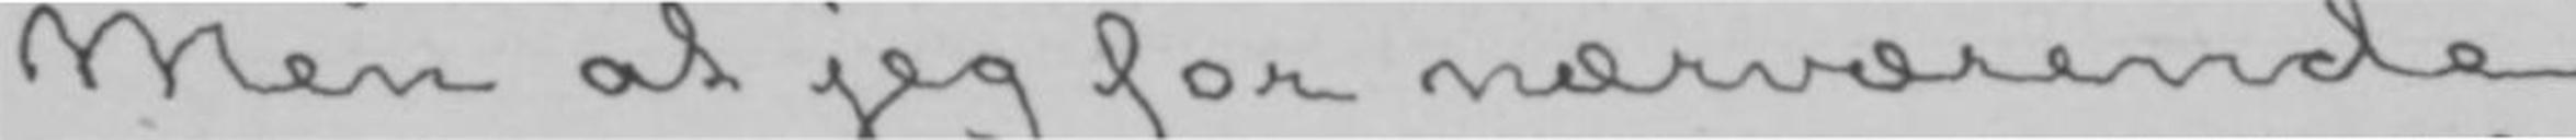Men at jeg for nærværende
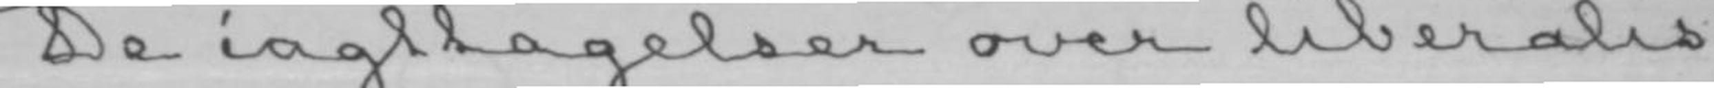De iagttagelser over liberalis-
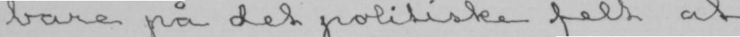bare på det politiske felt at
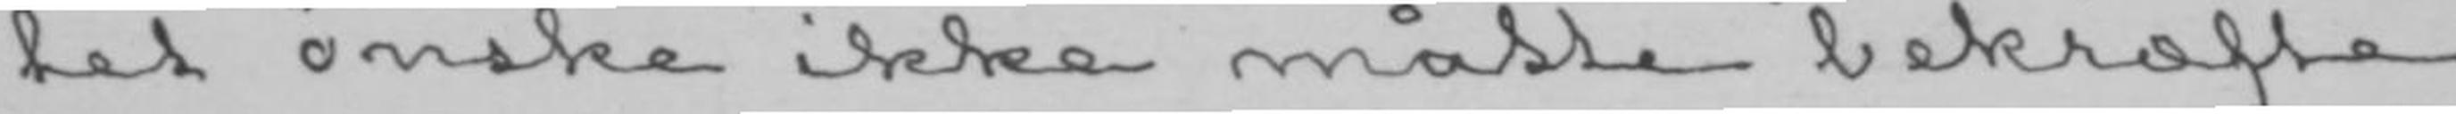tet ønske ikke måtte bekræfte
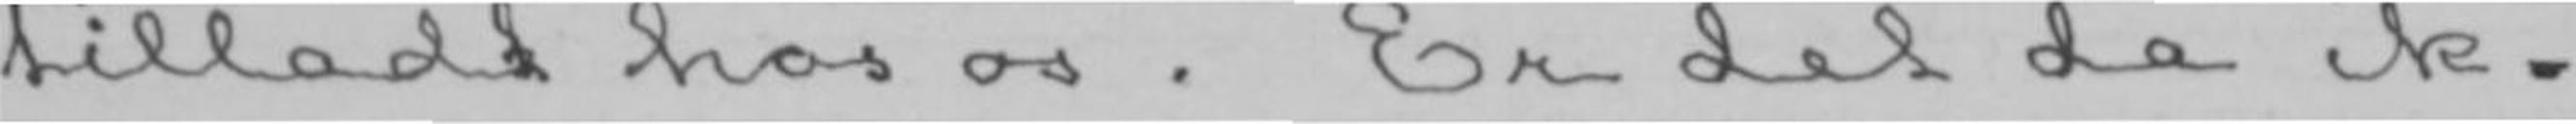tilladt hos os? Er det da ik-
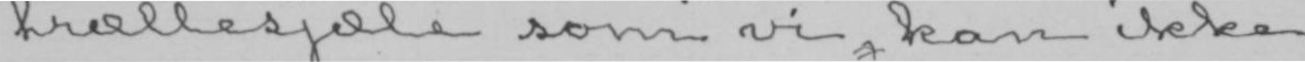trællesjæle som vi, kan ikke
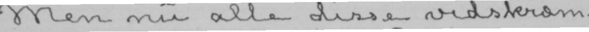Men nu alle disse vidskræm-
